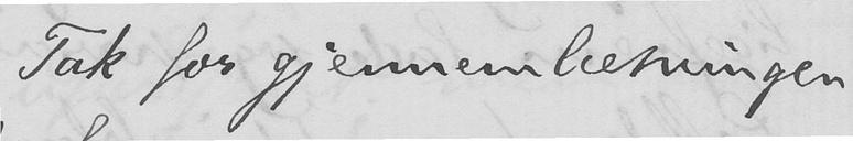Tak for gjennemlæsningen
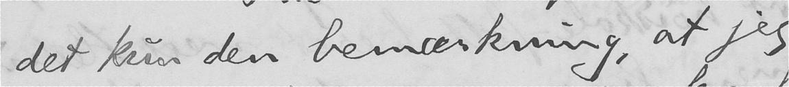det kun den bemærkning, at jeg
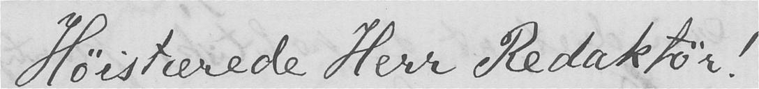Høistærede Herr Redaktør!
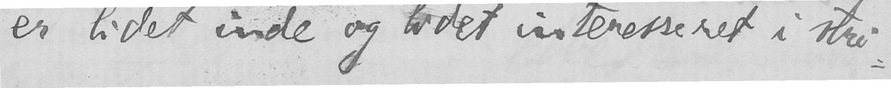er lidet inde og lidet interesseret i stri-
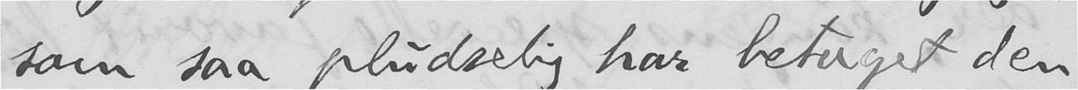som saa pludselig har betaget den
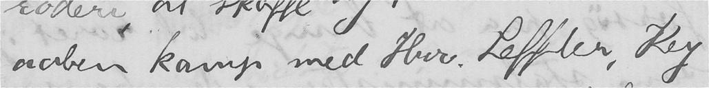aaben kamp med Hrr. Leffler, Key
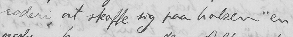roderi, at skaffe sig paa halsen "en
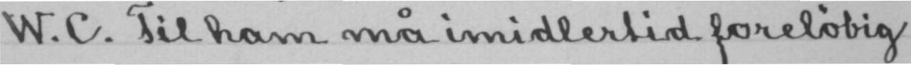W. C. Til ham må imidlertid foreløbig
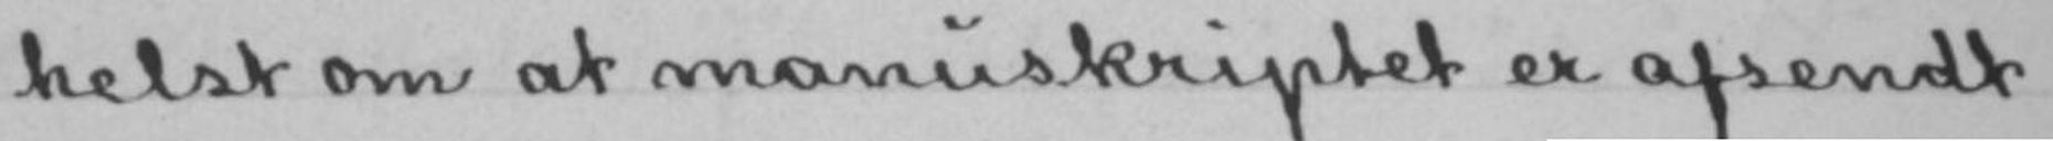helst om at manuskriptet er afsendt
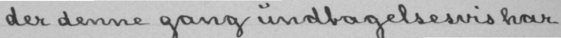der denne gang undtagelsesvis har
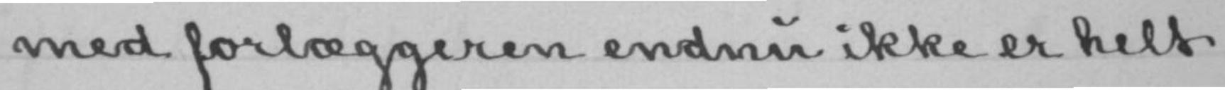med forlæggeren endnu ikke er helt
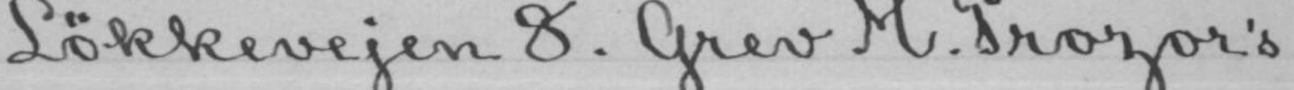Løkkevejen 8. Grev M. Prozor's
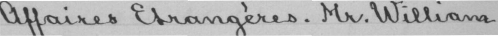Affaires Etrangères. Mr. William
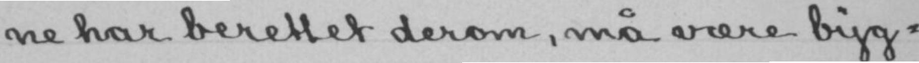ne har berettet derom, må være byg-
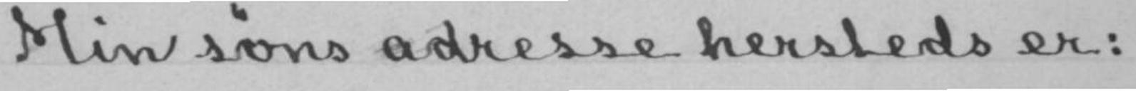Min søns adresse hersteds er:
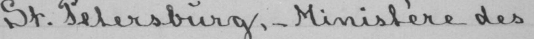St. Petersburg,- Ministère des
